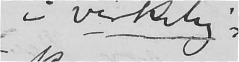i virkelig
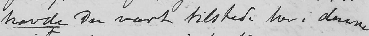havde Du vært tilstede her i denne
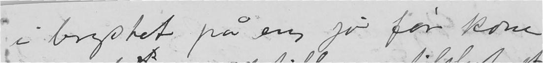i brystet på en, jo før kom-
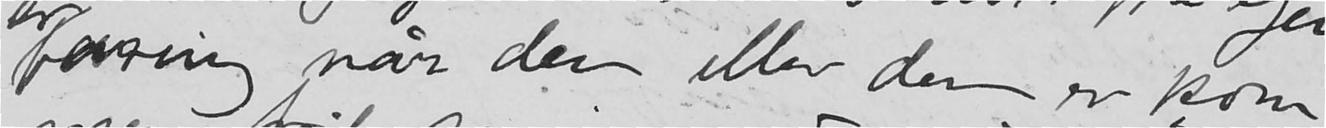faring når den eller den er kom
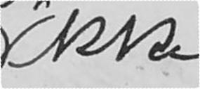ikke
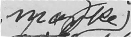mærke
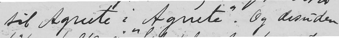til Agnete i "Agnete". Og desuden
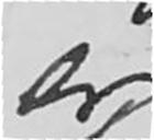er-
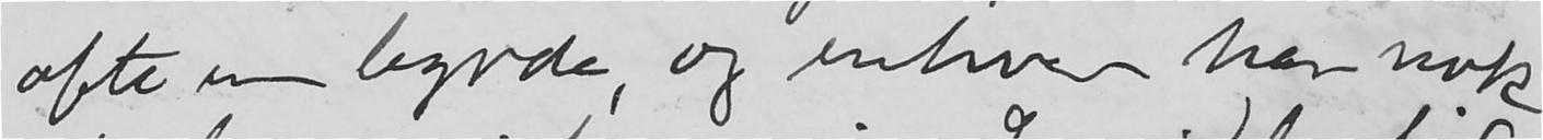ofte en byrde, og enhver har nok
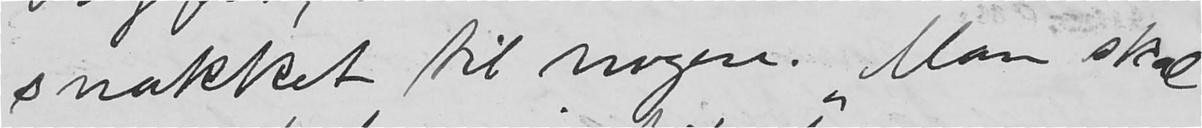snakket til nogen. "Man skal
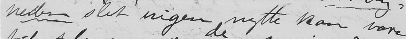heden slet ingen nytte kan være
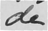de
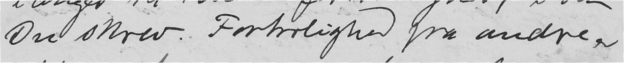Du skrev. Fortrolighed fra andre er
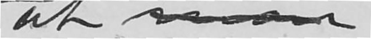at man
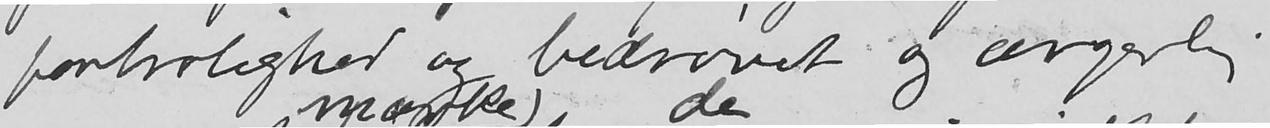fortrolighed og bedrøvet og ærgerlig
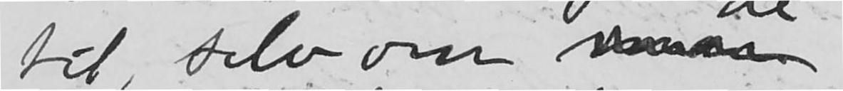til, selv om man
